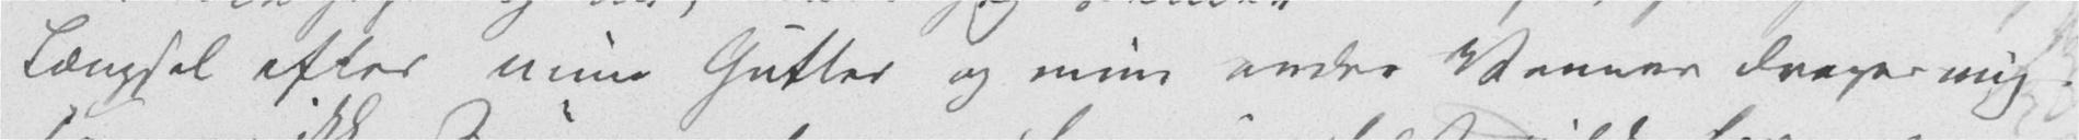Længsel efter mine Gutter og mine andre Venner drager mig.
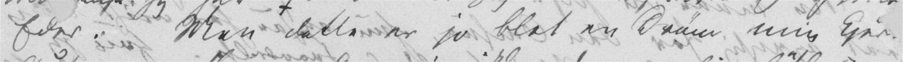Eder. Men dette er jo blot en Drøm min kjer
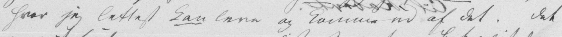hvor jeg lettest kan leve og komme ud af det. Det
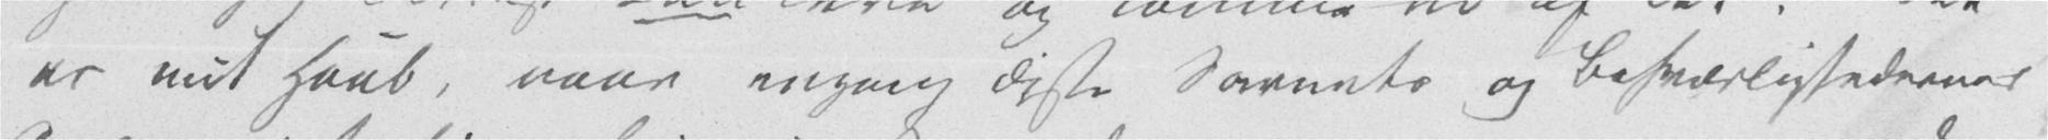er mit Haab, naar engang disse Savnets og Besværlighedernes
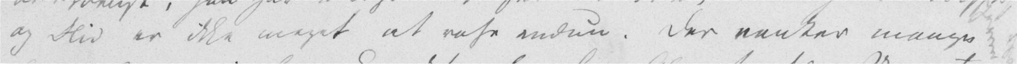og Flid er ikke meget at rose endnu. Der vanker mange
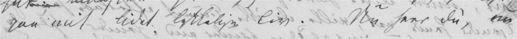paa mit lidet lykkelige Liv. Nu seer Du, nu
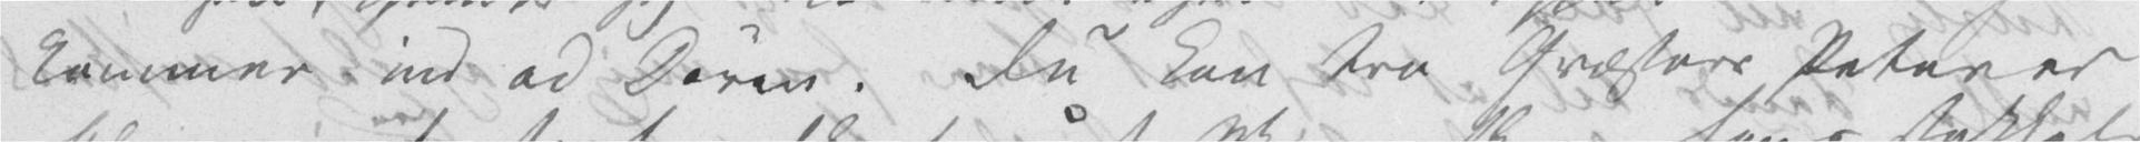kommer ind ad Døren. Du kan tro Qvæstors Peter er
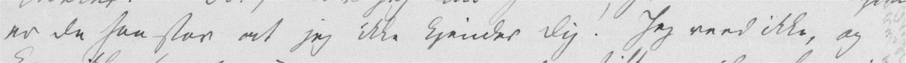er Du saa stor at jeg ikke kjender Dig! Jeg veed ikke, og
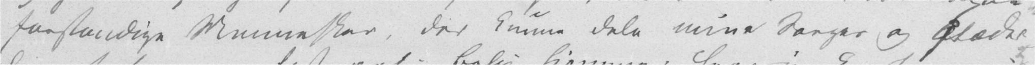forstandige Mennesker, der kunne dele mine Sorger og Glæder,
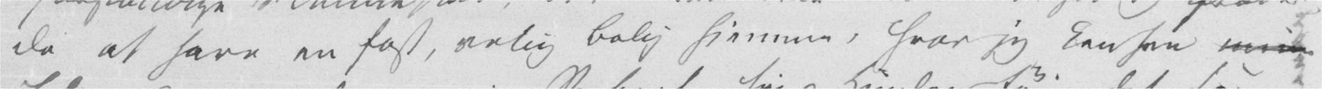da at have en fast, rolig Bolig hjemme, hvor jeg kan see mine
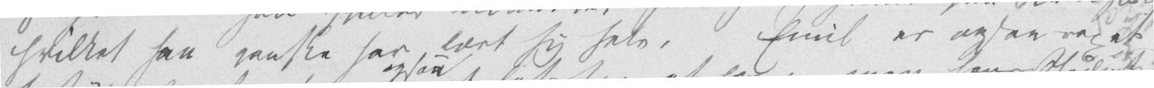hvilket han ganske har lært sig selv, Emil er ogsaa voxet
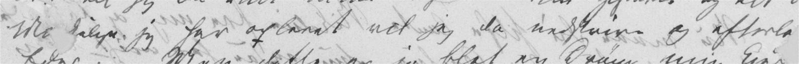Mærkelige jeg har oplevet vil jeg da nedskrive og efterla
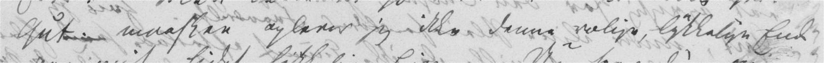Gut. maaske oplever jeg ikke denne rolige, lykkelige Ende
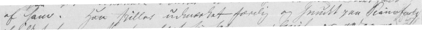af ham. Han spiller udmærket færdig og smukt paa Pianoforte
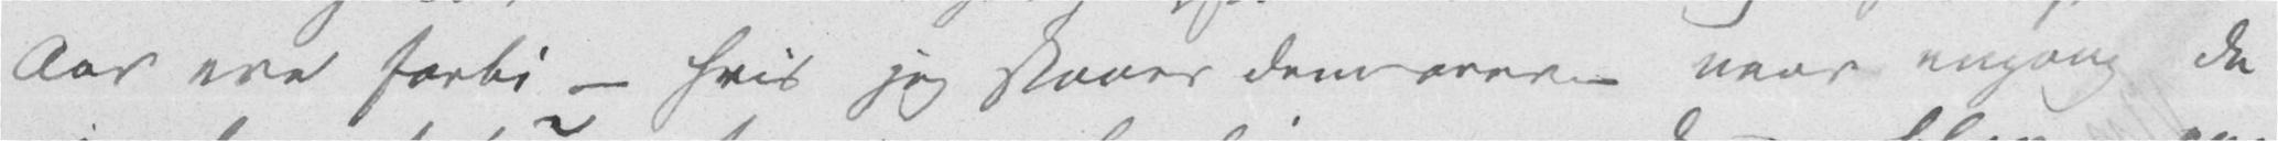Aar ere forbi – hvis jeg staaer dem over – naar engang de
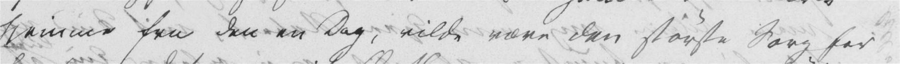hjemme fra den en Dag, vilde være den største Sorg for
